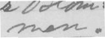men.
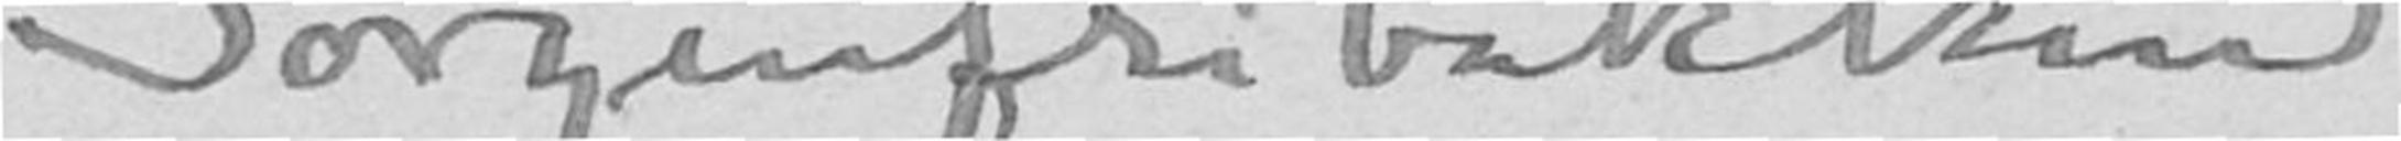Sorgenfribakken
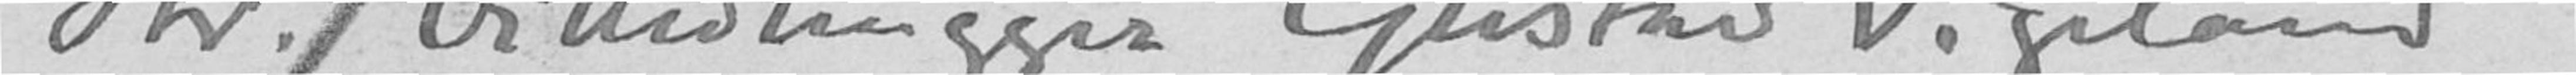Hr. billedhugger Gustav Vigeland
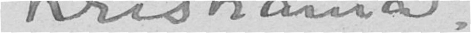Kristiania.
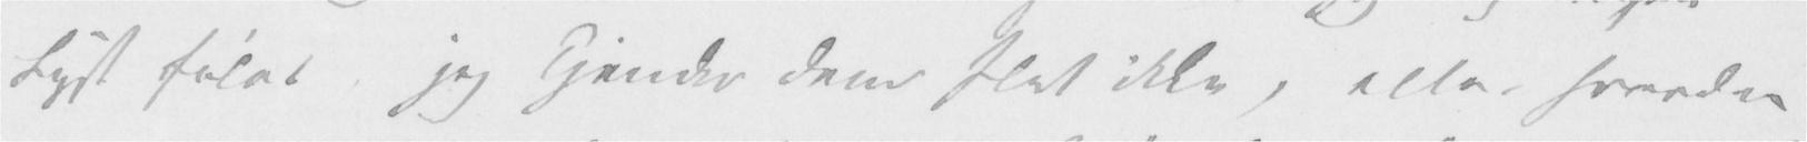Lyst føler, jeg kjender dem slet ikke, eller hvordan
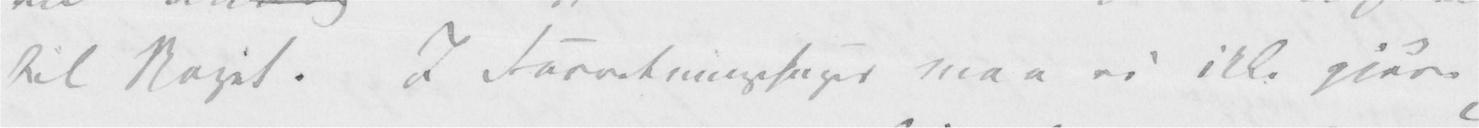til Noget. I Forretningssager maa vi ikke gjøre
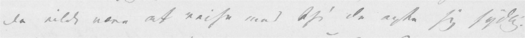de vilde være at reise med thi de agte sig sydlig.
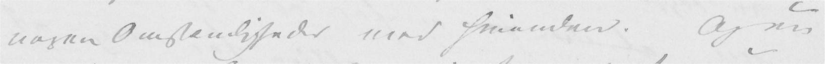nogen Omstændigheder mod hinanden. Og nu
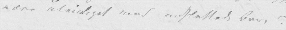være uleiliget med indsluttede Brev?
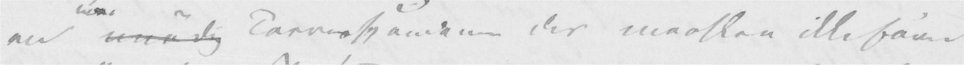en unødig Korresspondence der maaskee ikke fører
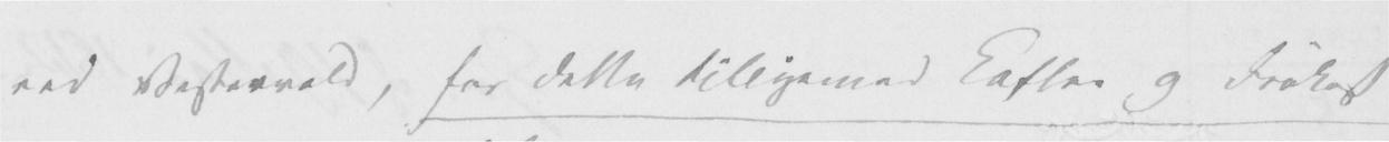ved Vestervold, for dette tiligemed Kaffee og Frokost
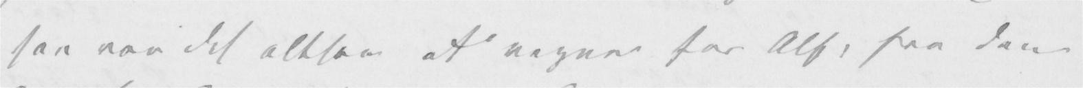saa var det altsaa at regne for Alf, fra den
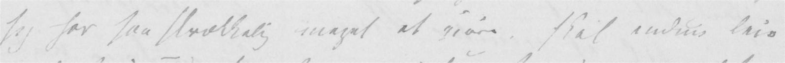Jeg har saa skrækkelig meget at gjøre. Skal endnu leie
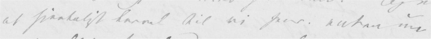et hjerteligt Levvel til vi sees. enten nu
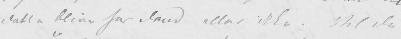dette bliver hos Dem eller ikke. Vil De
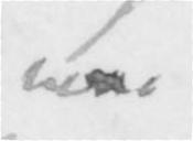<...>
